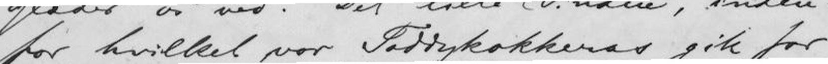for hvilket vor Toddykokkeras gik for
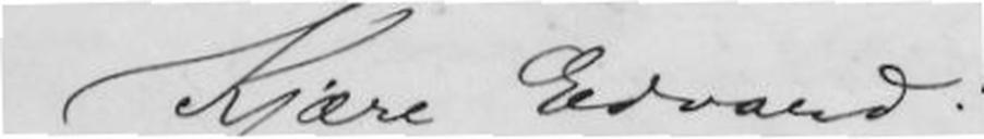Kjære Edvard!
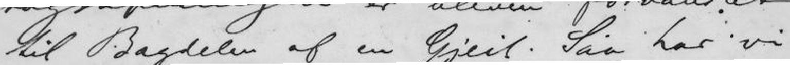til Bagdelen af en Gjeit. Saa har vi
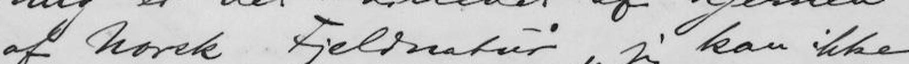af Norsk Fjeldnatur, jeg kan ikke
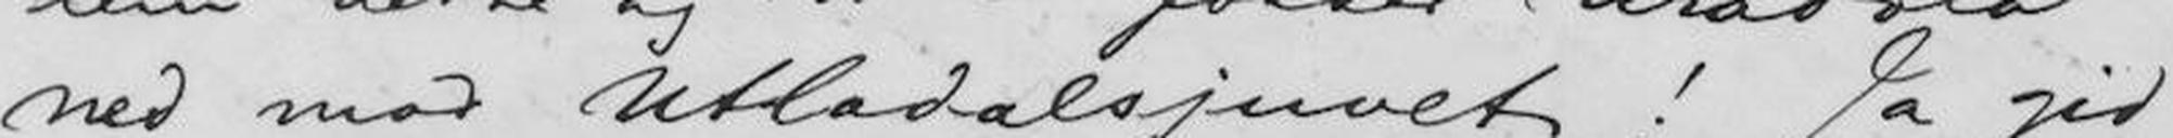ned mod Utladalsjuvet! Ja gid
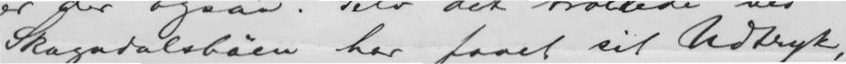Skogadalsbøen har faaet sit Udtryk,
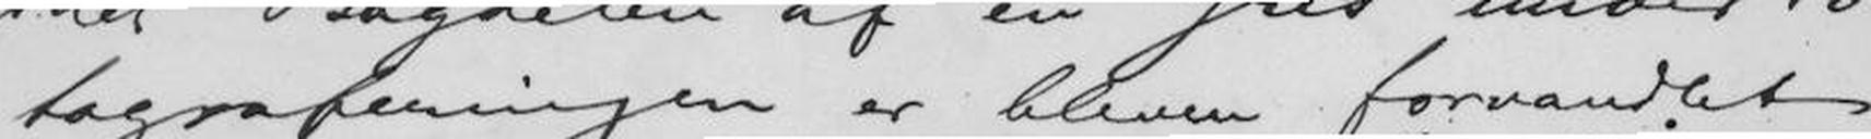tograferingen er bleven forvandlet
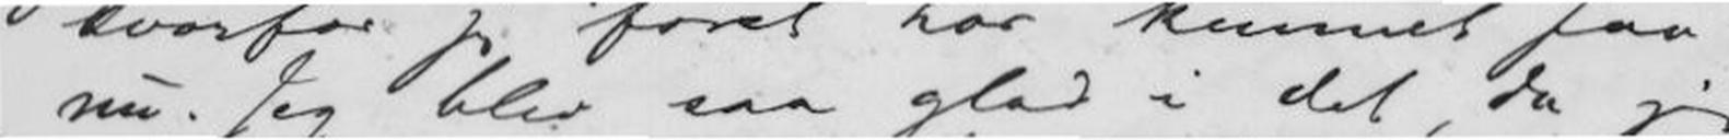nu. Jeg blev saa glad i det, da jeg
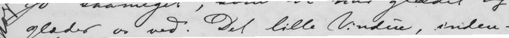glæder os ved. Det lille Vindue, inden-
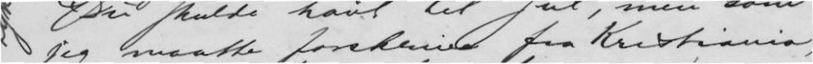jeg maatte forskrive fra Kristiania,
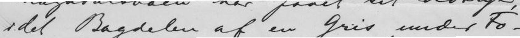idet Bagdelen af en Gris under Fo-
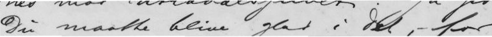Du maatte blive glad i det, -for
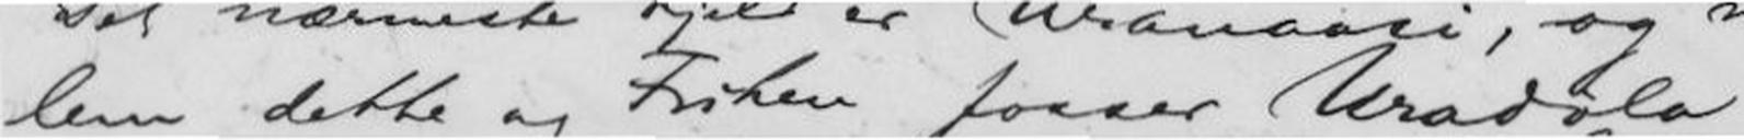lem dette og Friken fosser Uradøla
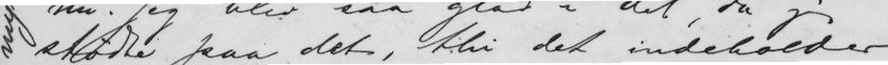stødte paa det, thi det indeholder
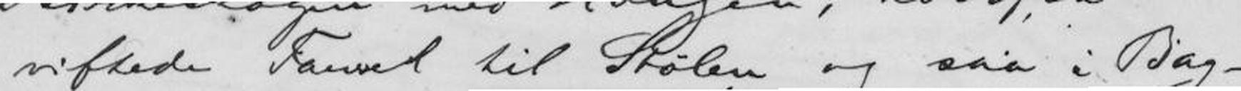viftede Farvel til Stølen og saa i Bag-
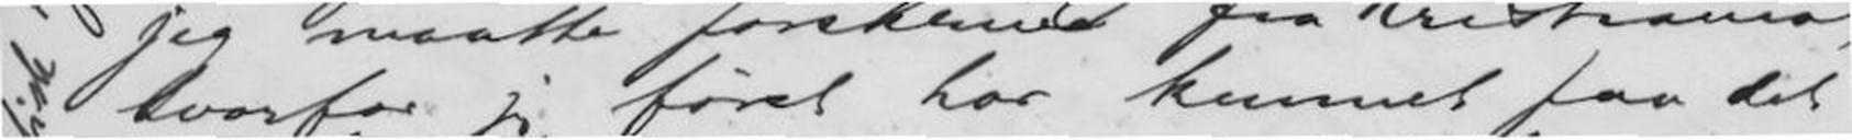hvorfor jeg først har kunnet faa det
